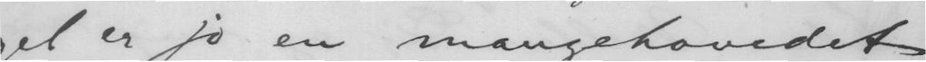get er jo en mangehovedet
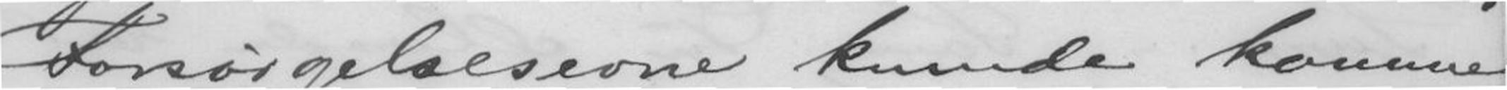Forsørgelsesevne kunde komme
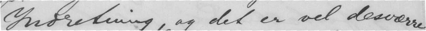Indretning, og det er vel desværre
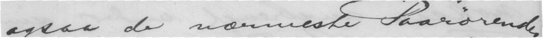ogsaa de nærmeste Paarørendes
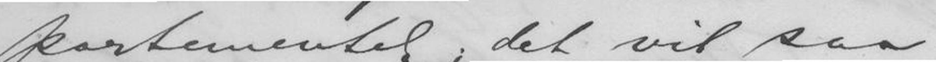partementet; det vil saa -
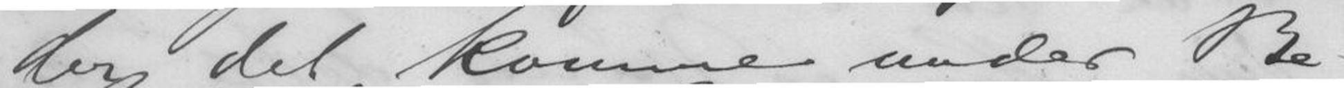der det, komme under Be-
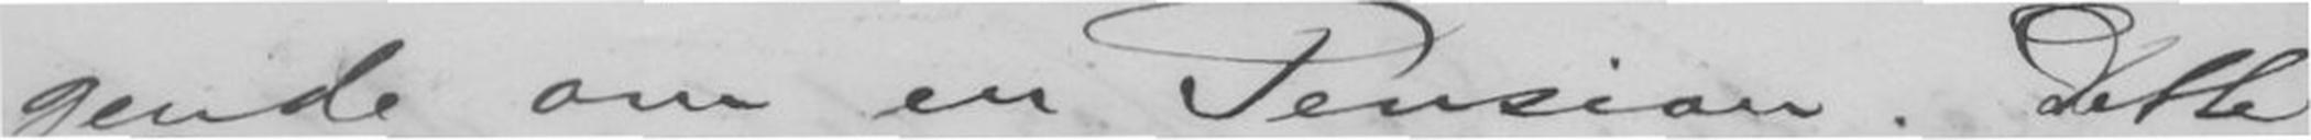gende om en Pension. Dette
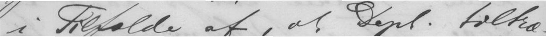i Tilfælde af, at Dept. tiltræ-
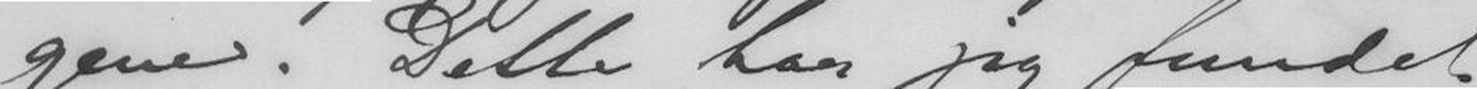gene. Dette har jeg fundet
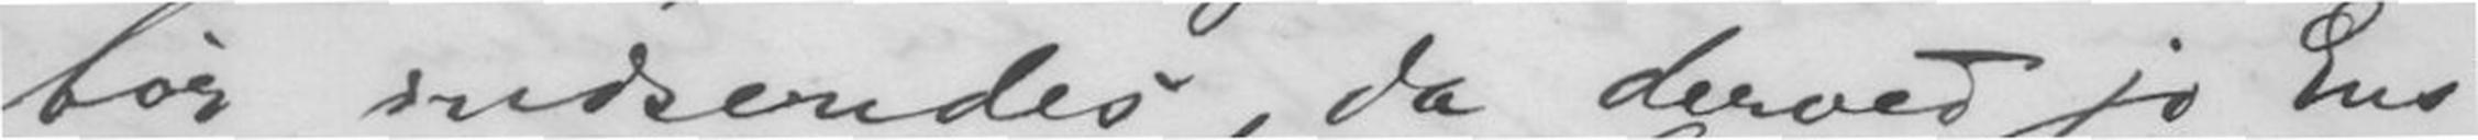bør indsendes, da derved jo Ens
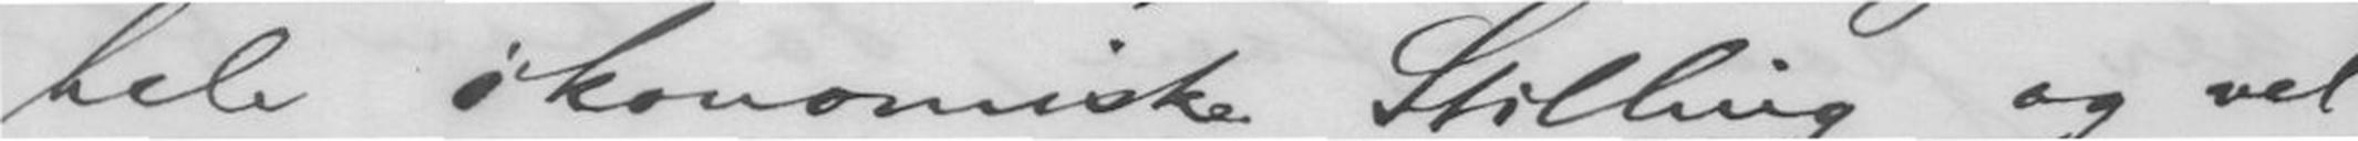hele økomomiske Stilling og vel
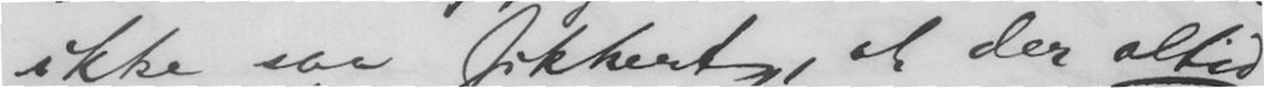ikke saa sikkert, at der altid
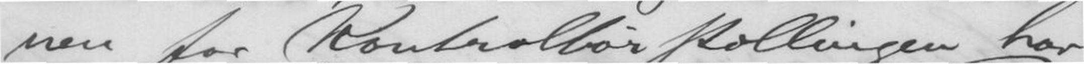nen for Kontrollørstillingen har
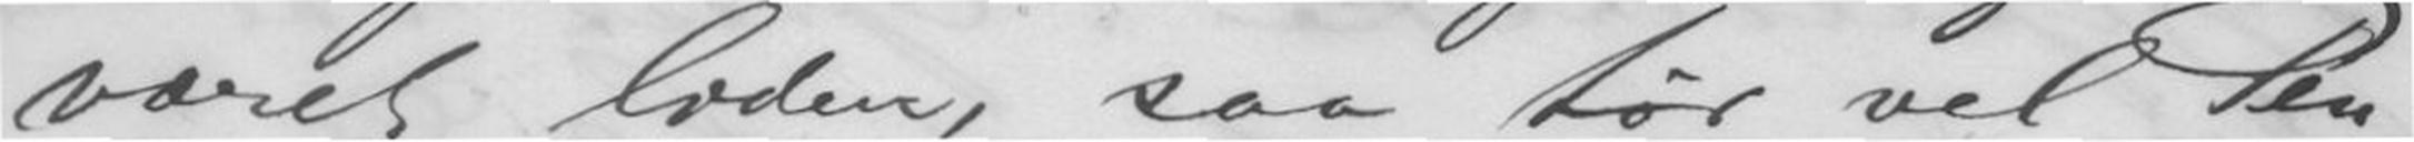været liden, saa tør vel Pen-
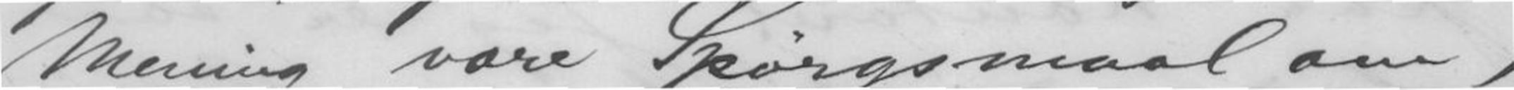Mening være Spørgsmaal om,
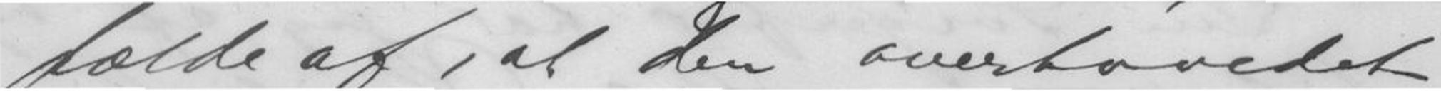fælde af, at den overhovedet
